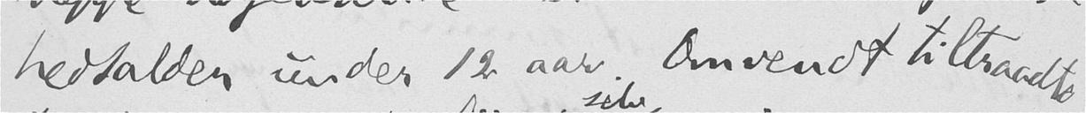hedsalder under 12 aar. Omvendt tiltraadte
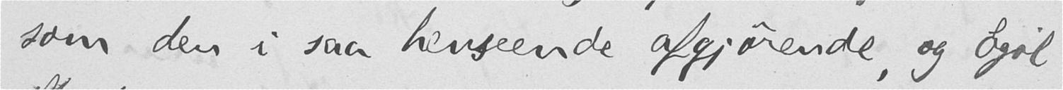som den i saa henseende afgjørende, og Egil
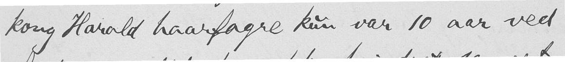kong Harald haarfagre kun var 10 aar ved
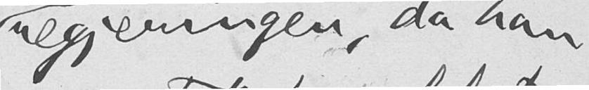regjeringen, da han
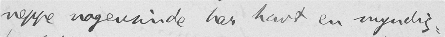neppe nogensinde har havt en myndig-
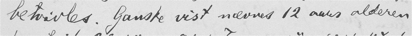betvivles. Ganske vist nævnes 12 aars alderen
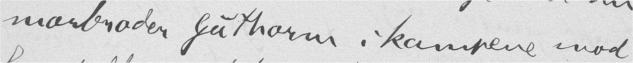morbroder Guthorm i kampene mod
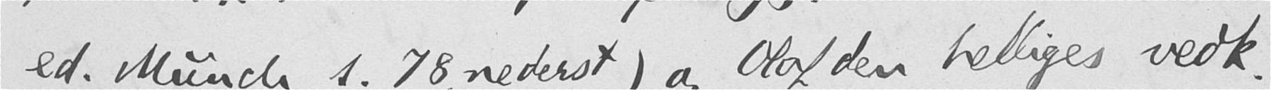ed. Munch s. 78, nederst) og Olaf den helliges vedk.
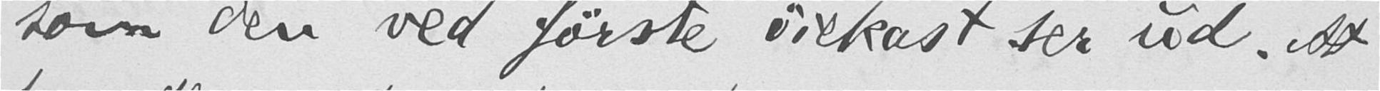som den ved første øiekast ser ud. At
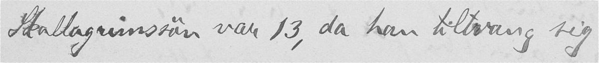Skallagrimssøn var 13, da han tiltvang sig
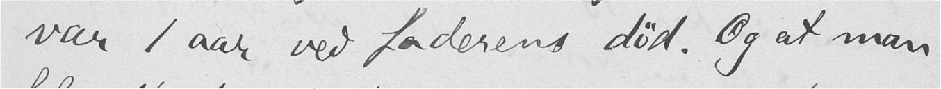var 1 aar ved faderens død. Og at man
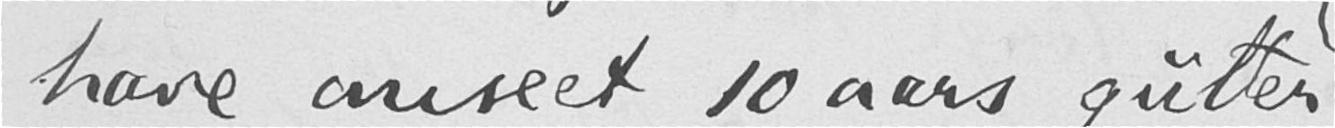have anseet 10 aars gutter
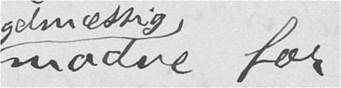modne for
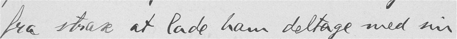fra strax at lade ham deltage med sin
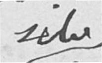selv
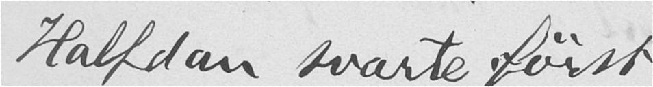Halfdan svarte først
